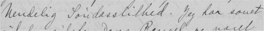Uendelig Søndasstilhed. Jeg har sovet
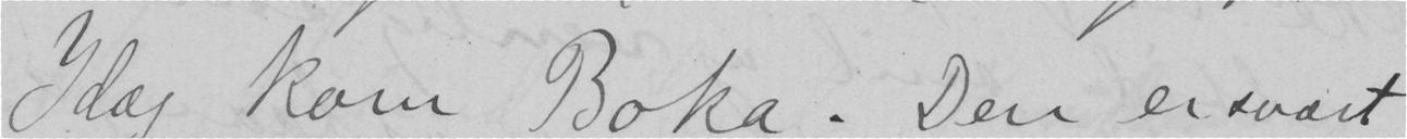Idag kom Boka. Den er svært
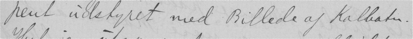pent udstyret med Billede af Kolbotn.
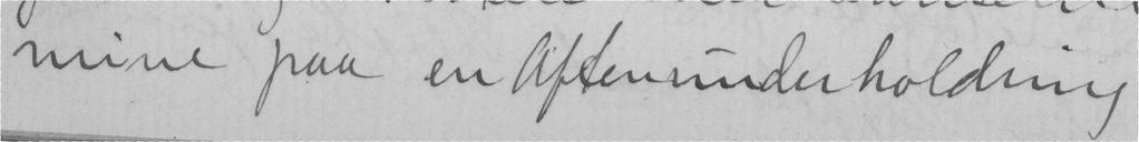mine paa en Aftenunderholdning
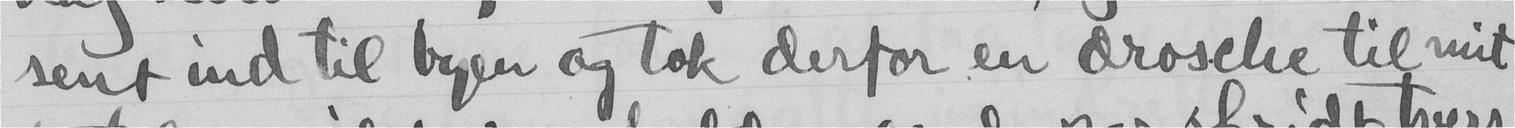sent ind til byen og tok derfor en drosche til mit
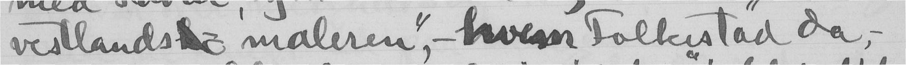vestlandske maleren", - hvem Folkestad da,-
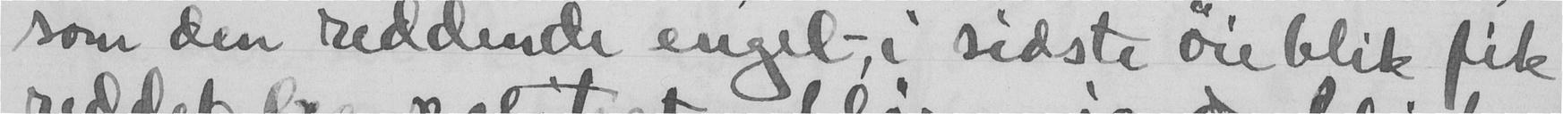som den reddende engel -, i sidste øieblik fik
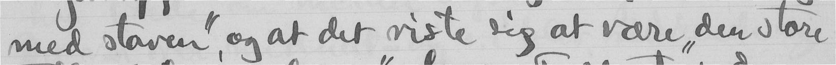med staven", og at det viste sig at være "den store
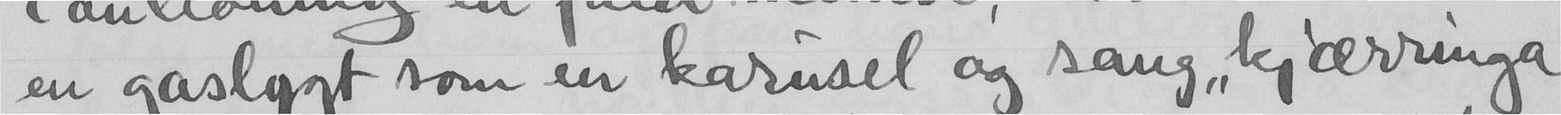en gaslygt som en karusel og sang "kjærringa"
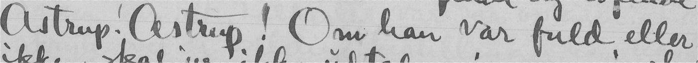Astrup! Astrup! Om han var fuld eller
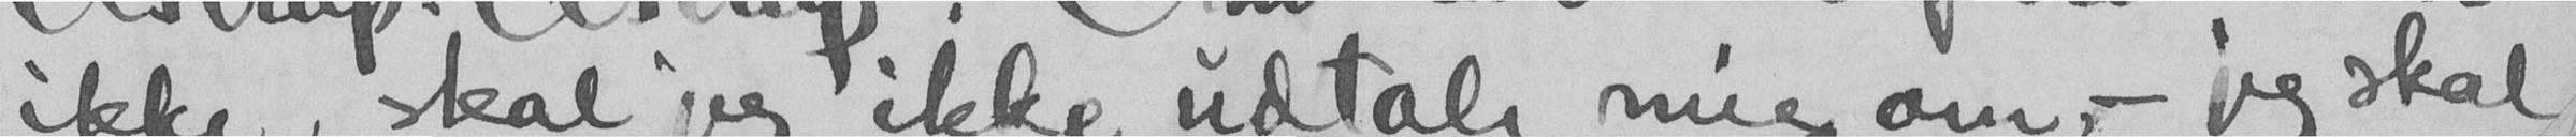ikke, skal jeg ikke udtale mig om, - jeg skal
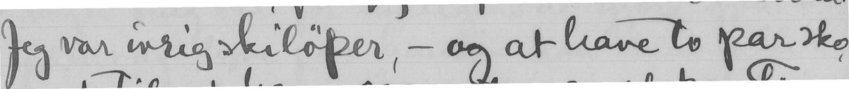Jeg var ivrig skiløper - og at have to par sko
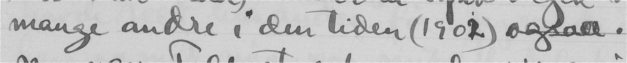mange andre i den tiden (1902) ogsaa.
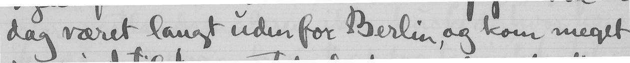dag været langt udenfor Berlin, og kom meget
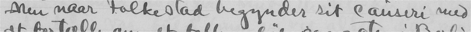Men naar Folkestad begynder sit Causeri med
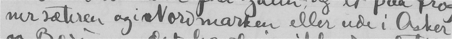nersæteren og Nordmarken eller ude i Asker
